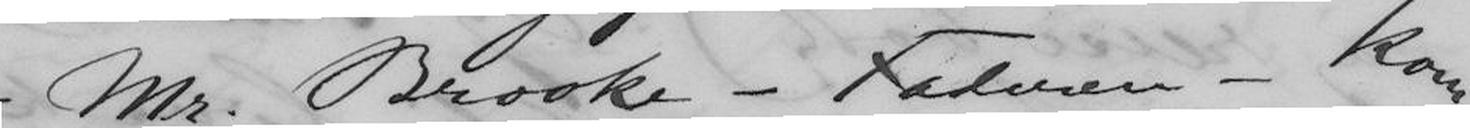- Mr. Brooke - Faderen - kom-
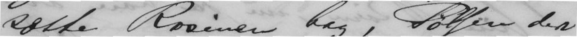sætte Rosinen bag, Pølsen den
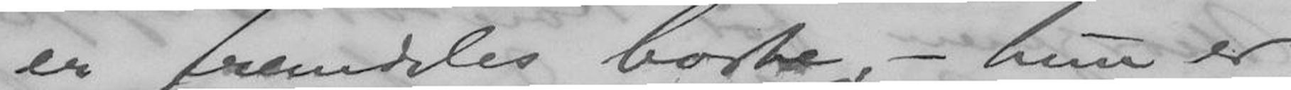er fremdeles borte - hun er
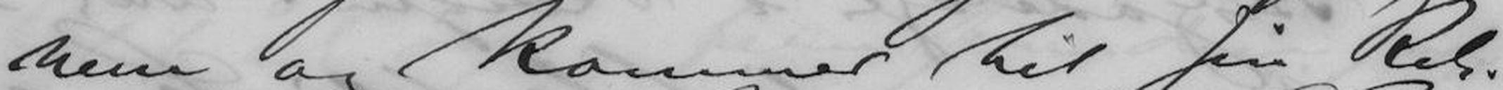nem og kommer til sin Ret.
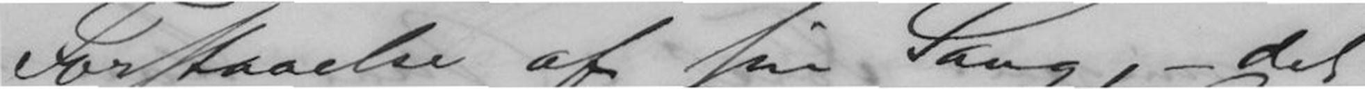Forstaaelse af sin Sang, - det
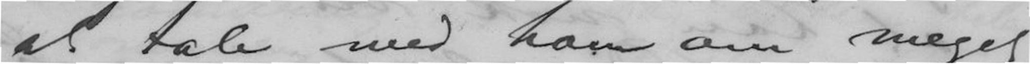at tale med ham om meget
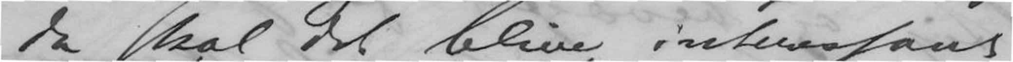da skal det blive interessant
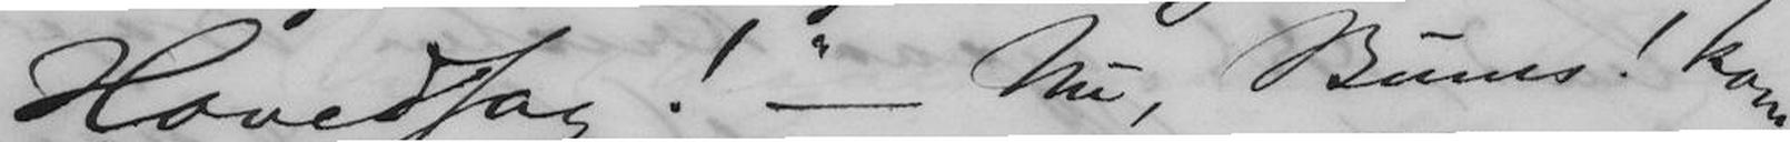Hovedsag!" - Nu, Bums! kom
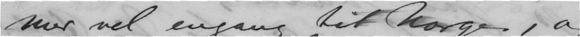mer vel engang til Norge, og
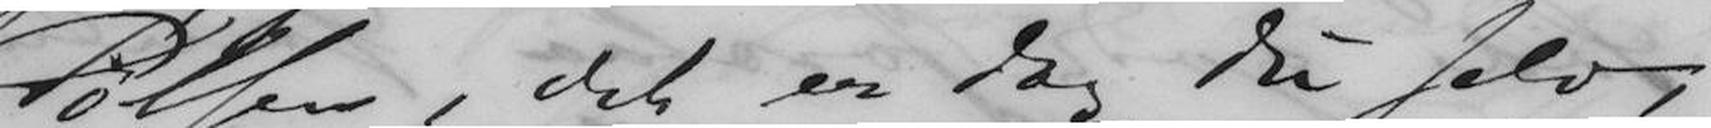Pølsen, det er dog Du selv, og
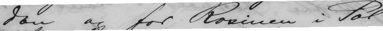don og for Rosinen i Pøl-
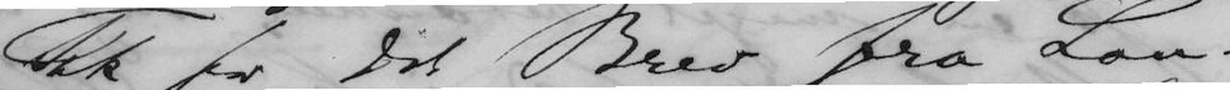Tak for Dit Brev fra Lon-
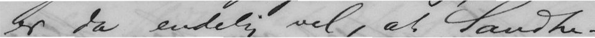er da endelig vel, at Sandhe-
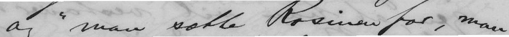"man sætte Rosinen for, man
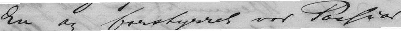En og forstyrret vor Passiar
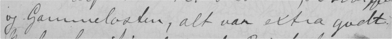og Gammelosten, alt var extra godt.
